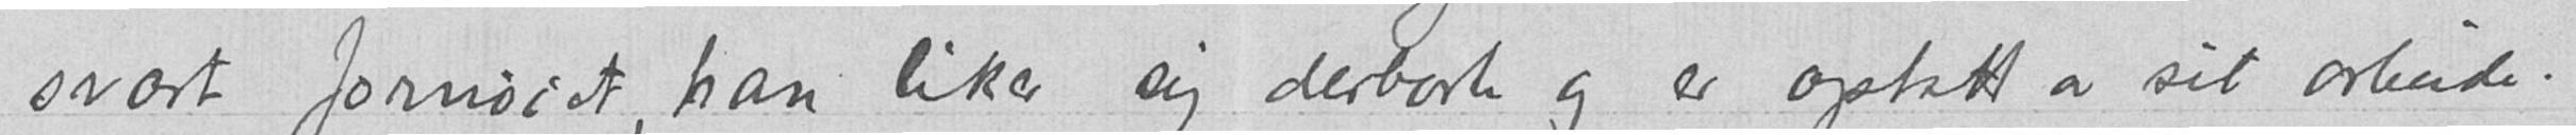svært fornøiet, han liker sig derborte og er optatt av sit arbeide.
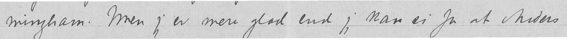mingham. Men jeg er mere glad end jeg kan si for at Anders
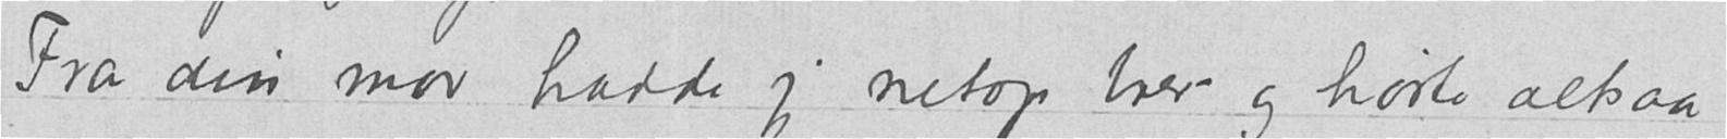Fra din mor hadde jeg netop brev og hørte altsaa
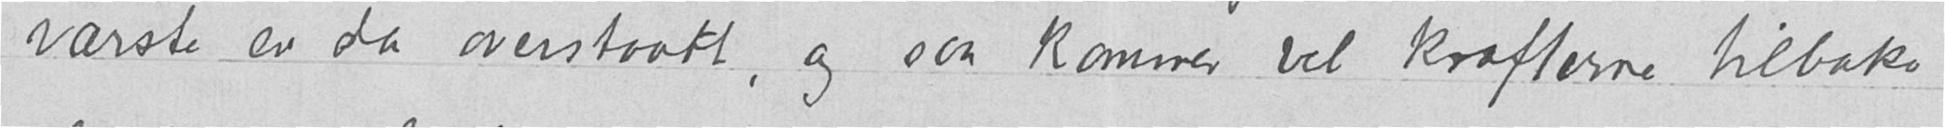værste er da overstaatt, og saa kommer vel kræfterne tilbake
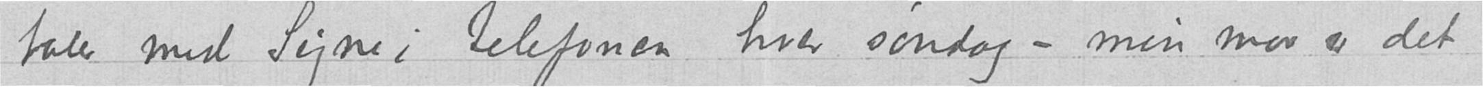taler med Signe i telefonen hver søndag – min mor er det
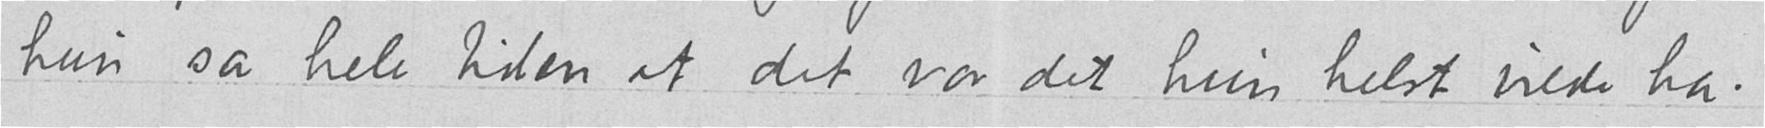hun sa hele tiden at det var det hun helst vilde ha.
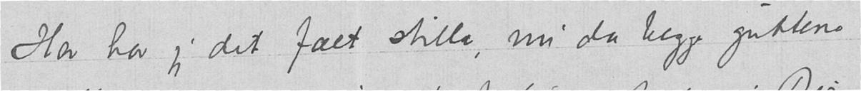Her har jeg det fælt stille, nu da begge guttene
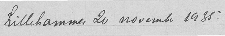Lillehammer 20 november 1935.
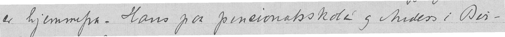er hjemmefra – Hans paa pensionatsskolen og Anders i Bir-
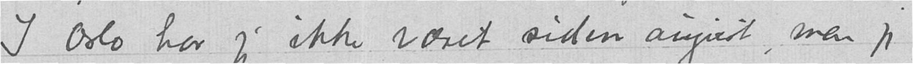I Oslo har jeg ikke været siden august, men jeg
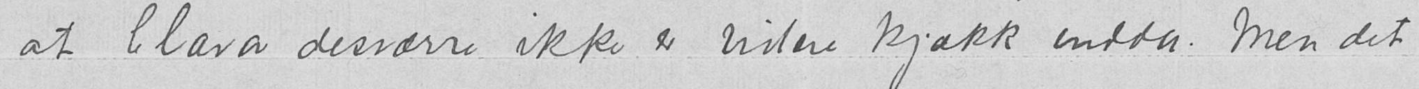at Clara desværre ikke er videre kjækk endda. Men det
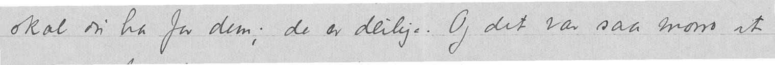skal du ha for dem; de er deilige. Og det var saa morro at
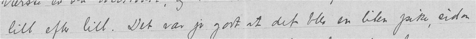litt efter litt. Det var jo godt at det blev en liten pike, siden
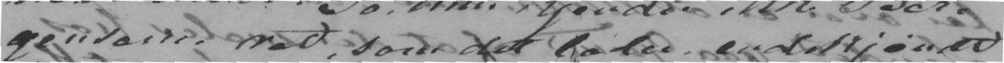genserne ret, som det lader endskjøndt
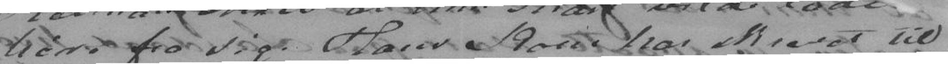høre fra sig. Hans Kone har skrevet til
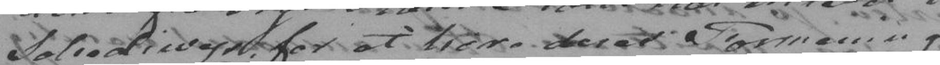Schediwys for at høre deres Formening
